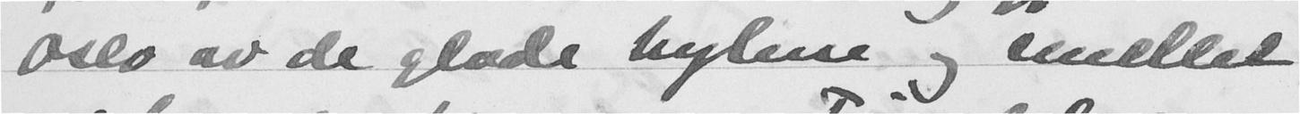Oslo av de glade hylene og smellet
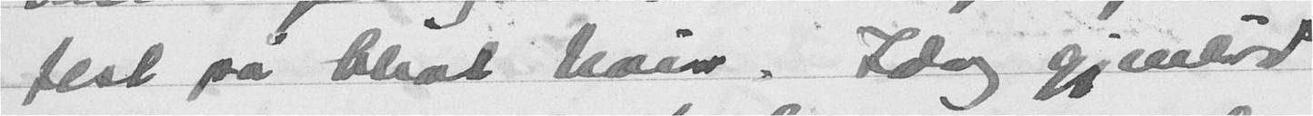fest på Khot leir. Idag gjenlød
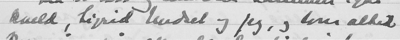kveld, Sigrid Undset og jeg, og som altid
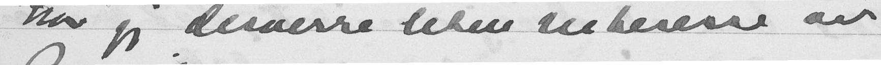har jeg desverre liten interesse av
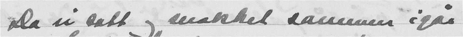Da vi satt og snakket sammen igår
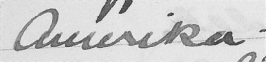Amerika.
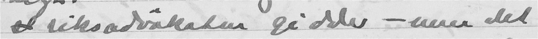riksadvokaten gidder - men det
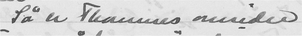Så er Thommes omsider
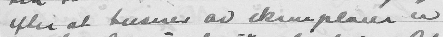efter at tusner av eksemplarer er
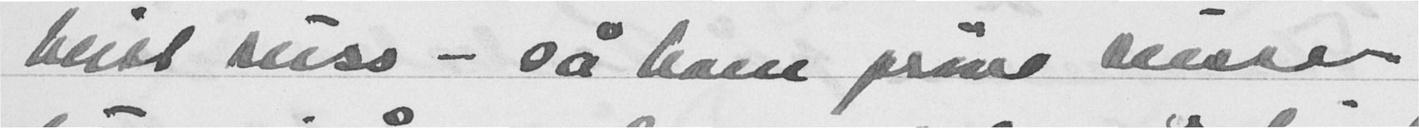blitt russ - så ham prøve russe-
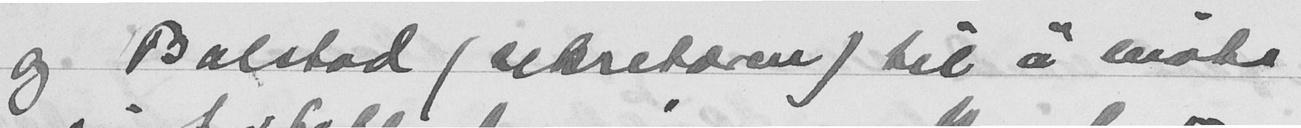Og Balstad (sekretæren) til å møte
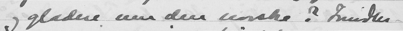og gladere enn den norske? Imidler-
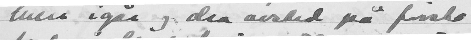luer igår og dra avsted på første
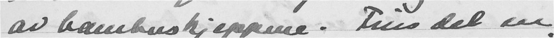av bambuskjeppene. Fins det en
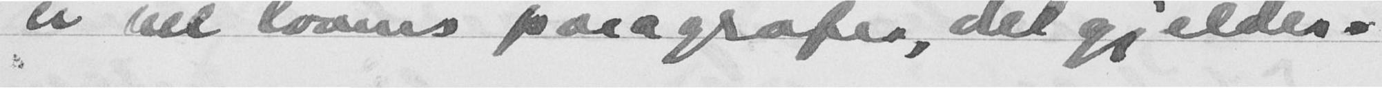er vel lovens paragrafer, det gjelder.
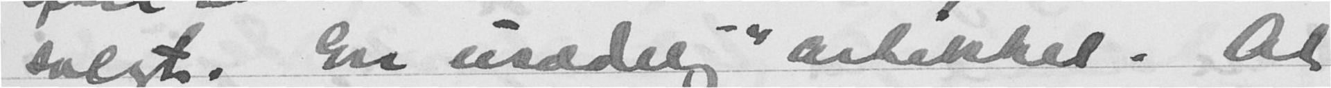solgt. En underlig artikkel. At
